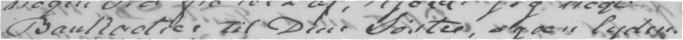Bankactier til Dine Søstre, og een lyden-
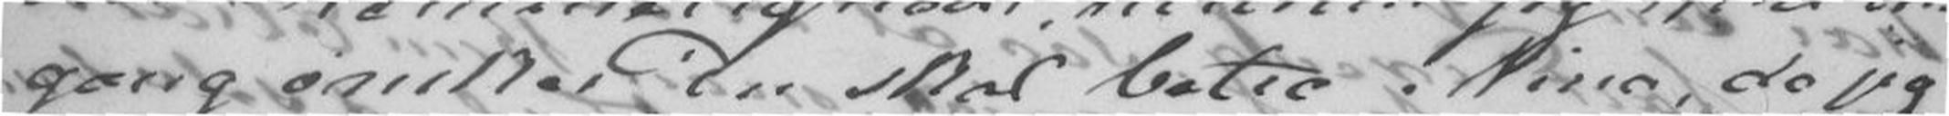gang ønsker Du skal betro Nina, da jeg
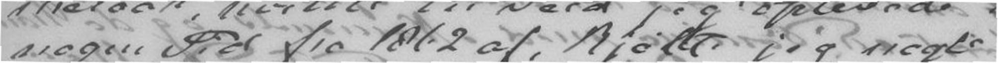nogen tid fra 1862 af, kjøbte jeg nogle
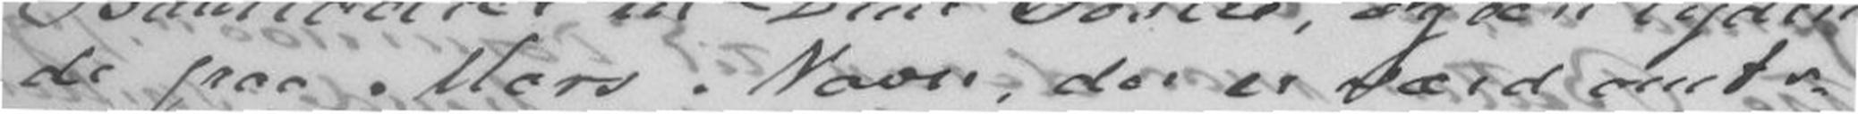de paa Mors Navn, der er værd omtr.
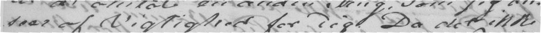seer af Vigtighed for Dig. da det ikke
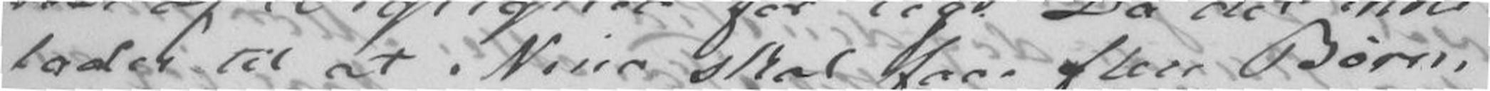lader til at Nina skal faae flere Børn,
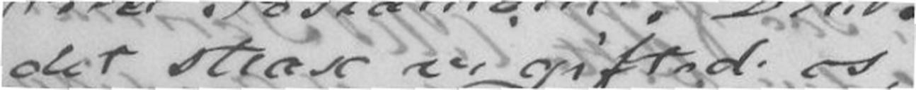det strax vi giftede os,
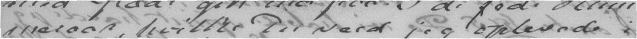meraar, hvilke Du veed jeg oplevede i
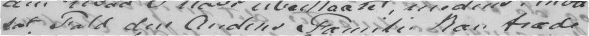sat Fald den Andens Familie kan træde
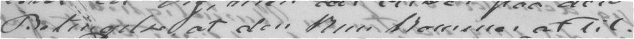Betingelse at den kun kommer at til-
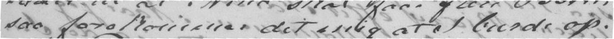saa forekommer det mig at I burde op-
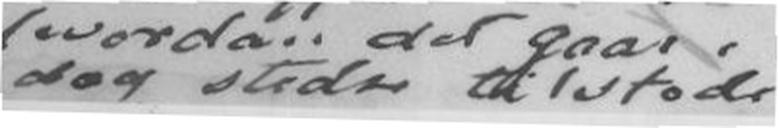dog stedse til stede.
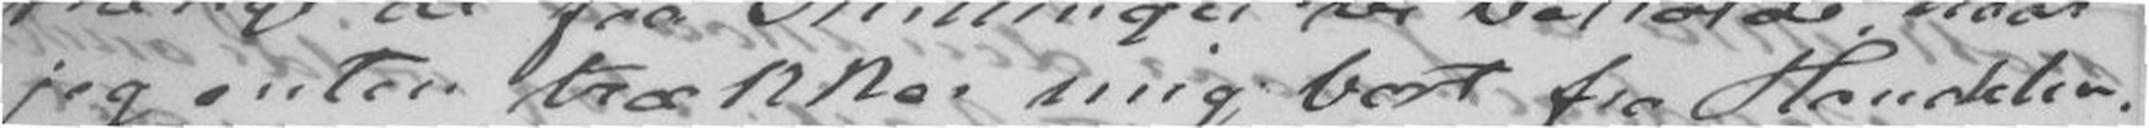jeg enten trækker mig bort fra Handelen,
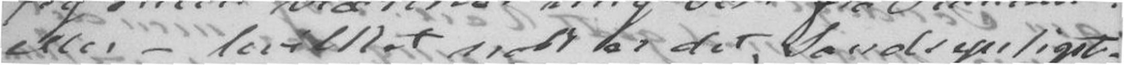eller - hvilket nok er det Sandsynligste
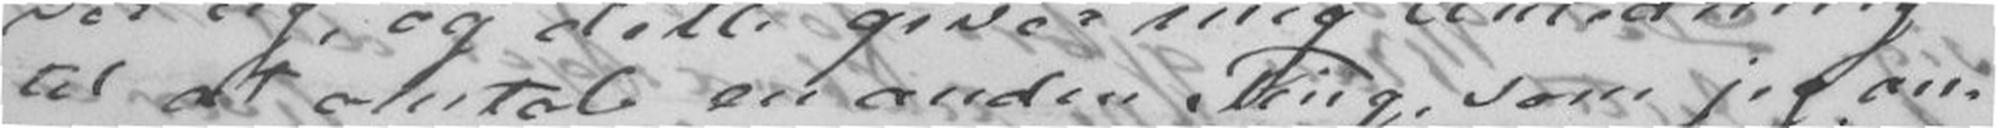til at omtale en anden Tiing, som jeg an-
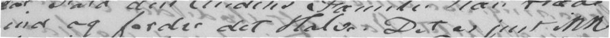ind og forder det Halve. Det er just ikke
